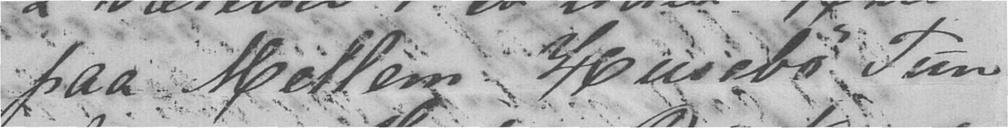paa Mellem-Husebø Tun
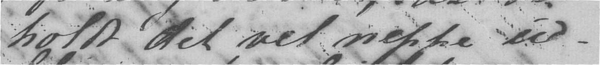holdt det vel neppe ud.
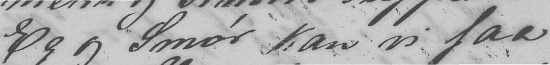Eg og Smør kan vi faa
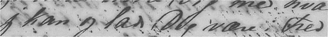jeg kan og lade Dig være i Fred
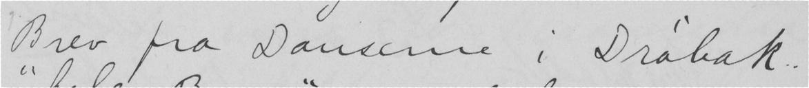Brev fra Danserne i Drøbak.
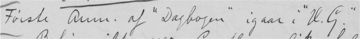Første Anm. af "Dagbogen" igaar i "V. G."
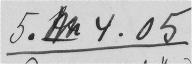5. 4.05
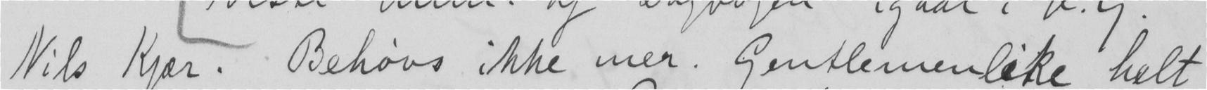Nils Kjær. Behøvs ikke mer. Gentlemenlike helt
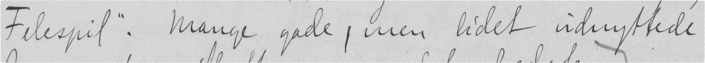Felespil". Mange gode, men lidet udnyttede
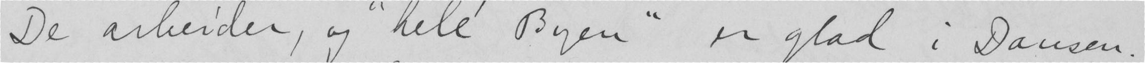De arbeider, og "hele Byen" er glad i Dansen.
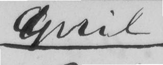April
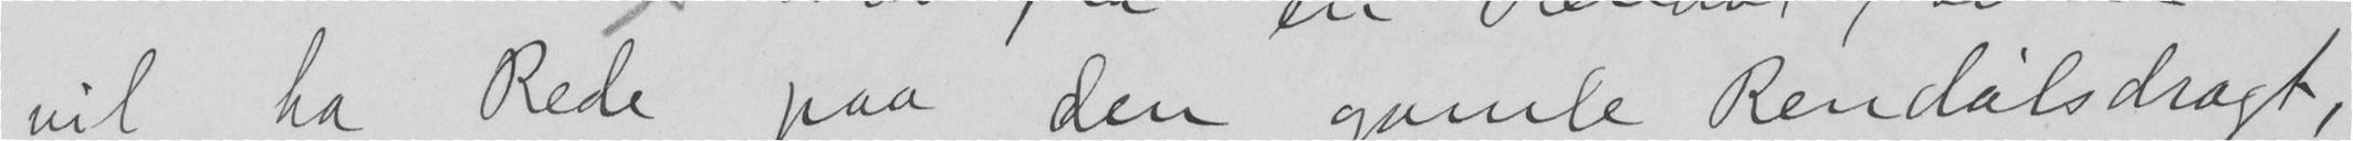vil ha Rede paa den gamle Rendalsdragt,
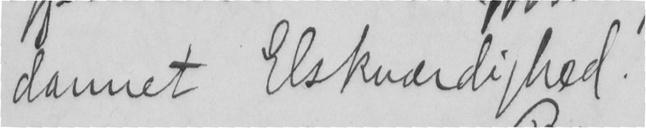dannet Elskværdighed.
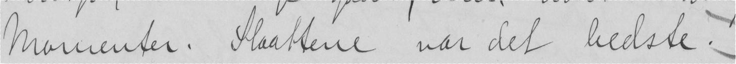Momenter. Slaattene var det bedste—
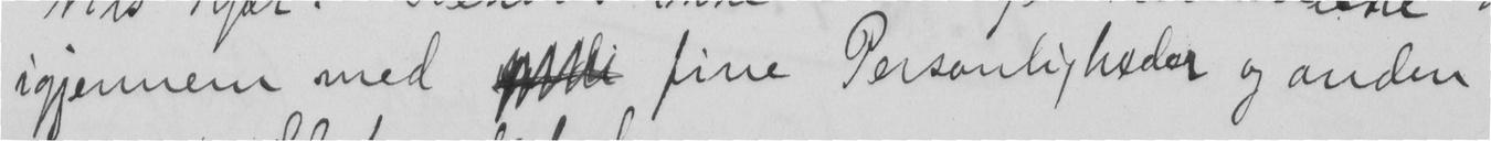igjennem med  fine Personligheder og anden
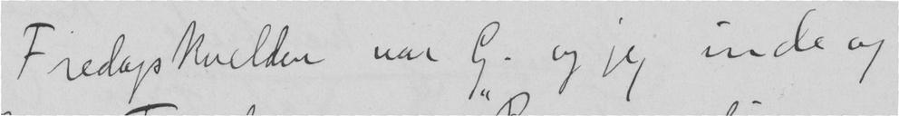Fredagskvelden var G. og jeg inde og
